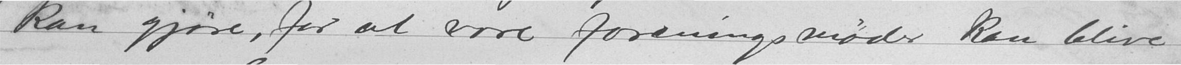kan gjøre, for at vore foreningsmøder kan blive
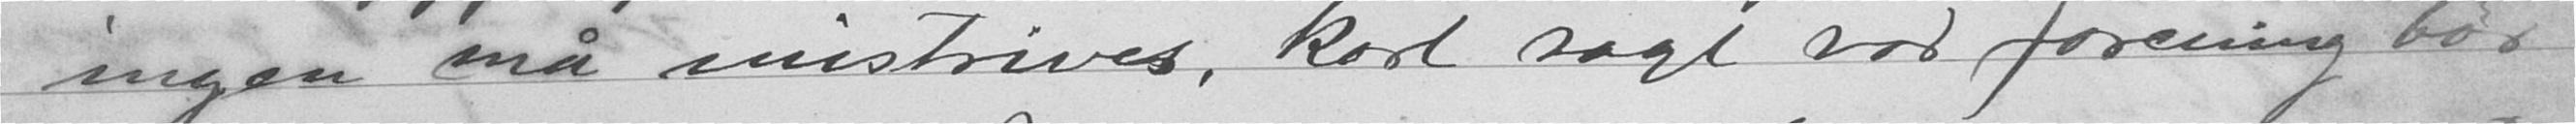ingen må mistrives, kort sagt vor forening bør
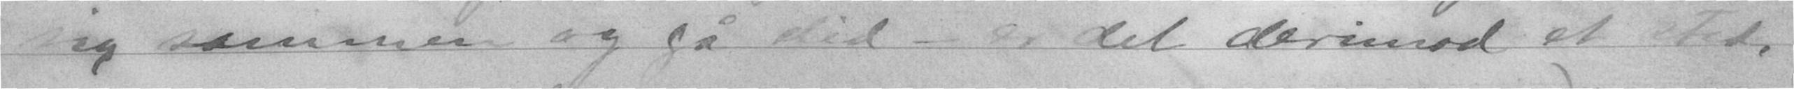sig sammen og gå did - er det derimot et et sted,
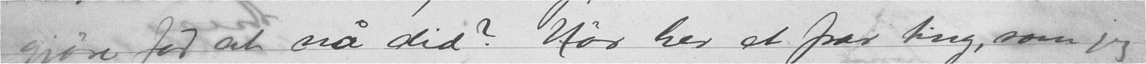gjøre for at nå did? Hør her et par ting, som jeg
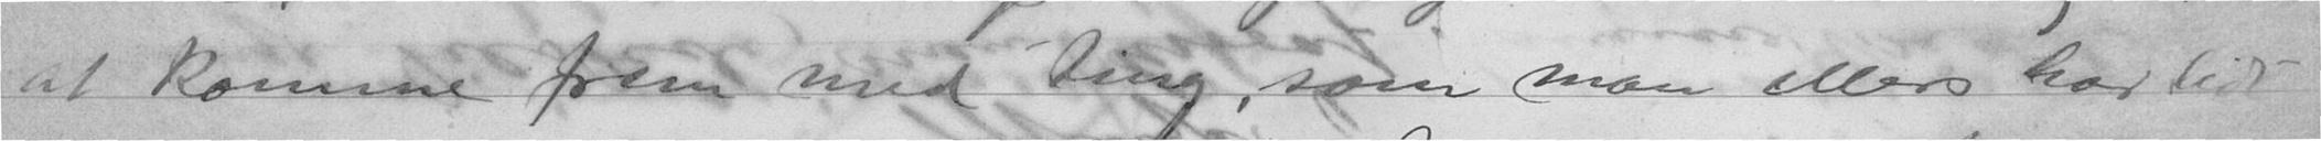at komme frem med ting, som man ellers har til-
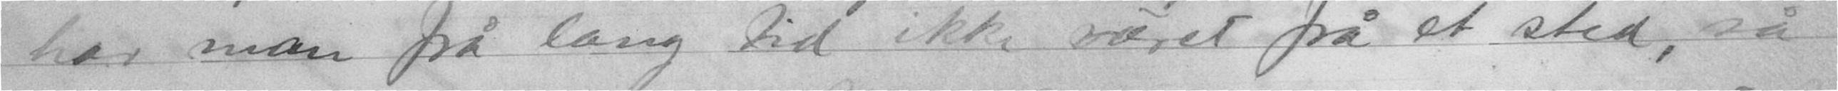har man på lang tid ikke været på et sted, så
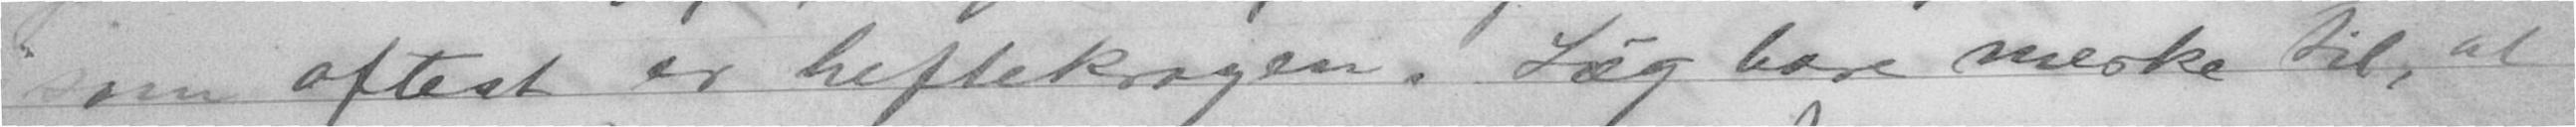som oftest er heftekragen. Læg bare merke til, at
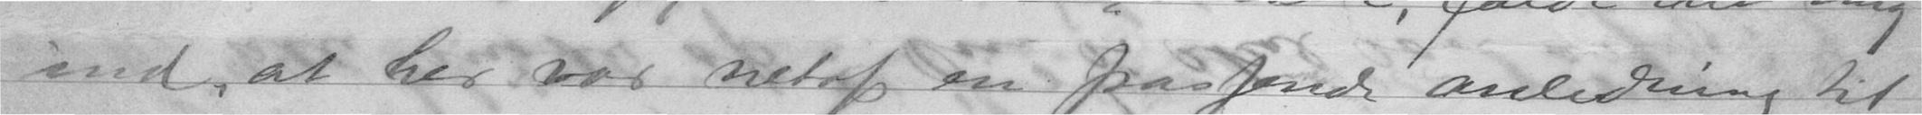ind, at her var i netop en paa jendr anendning til
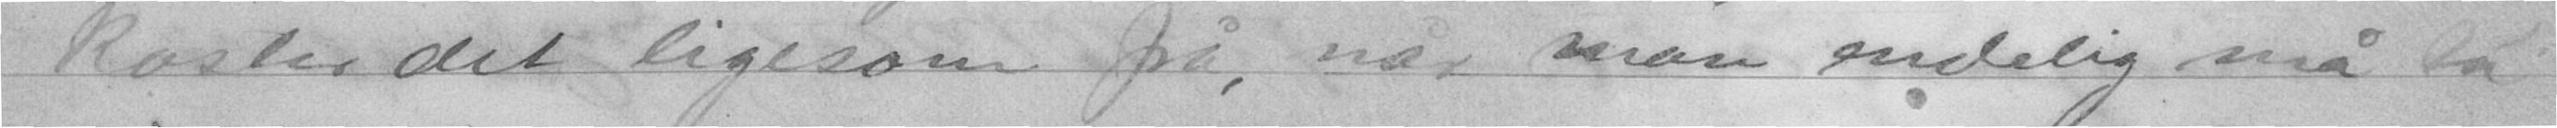koster det ligesom på når man endelig må ta
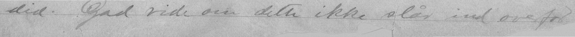did. Gud vide om dette ikke slår in overfor
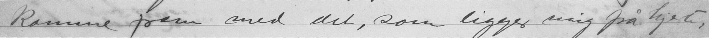komme frem med det, som ligger mig på hjerte,
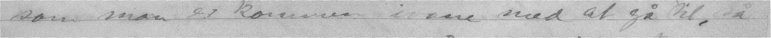som man er kommen i vane med at gå til, så
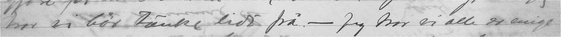tror vi bør tenke lidt på - Jeg tror vi alle er enige
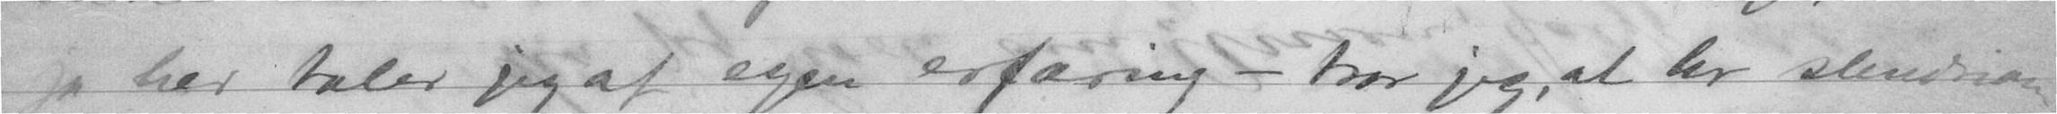ja her taler jeg af egen erfaring - tror jeg, at her slendsian
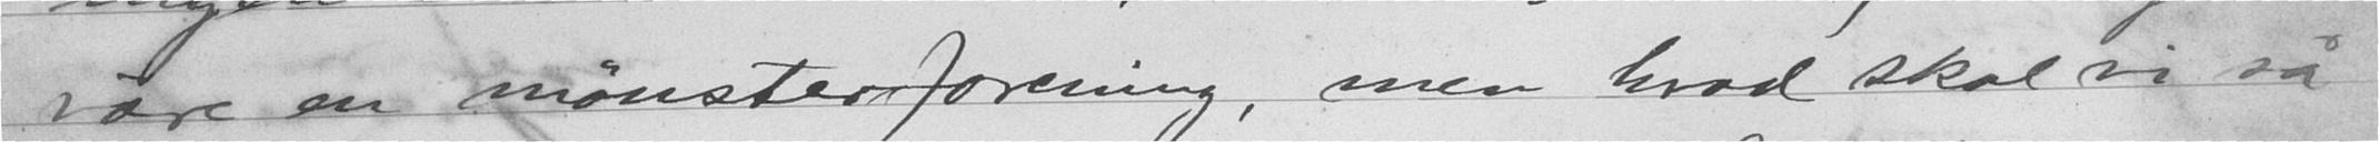være en mønsterforening, men hvad skal vi så
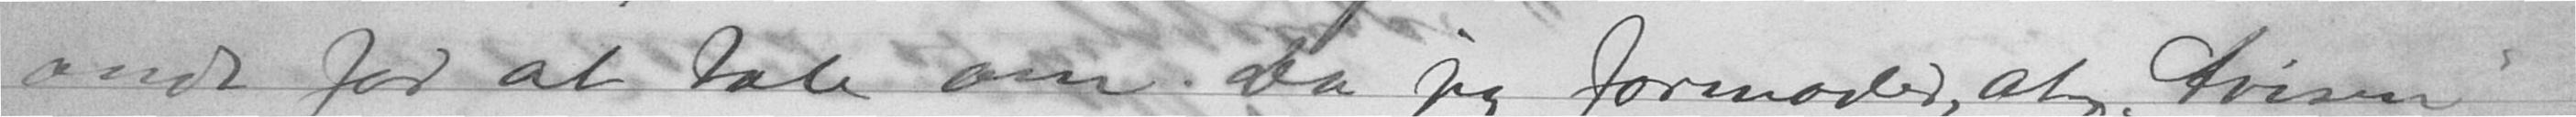mod for at tale om. da jeg formoder, at, trimen
